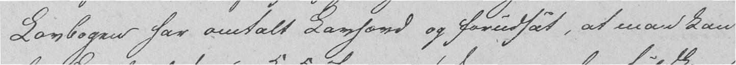Lovbogen har omtalt Lovhævd og forudsat, at man kan
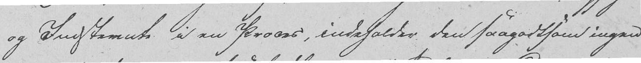og Indstevnte i en Proces, indeholder den saagodtsom ingen
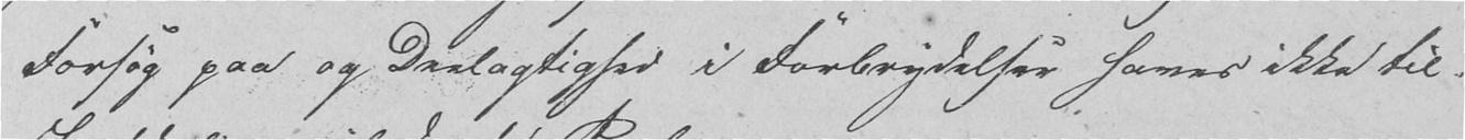Forsøg paa og Deelagtighed i Forbrydelser haves ikke til-
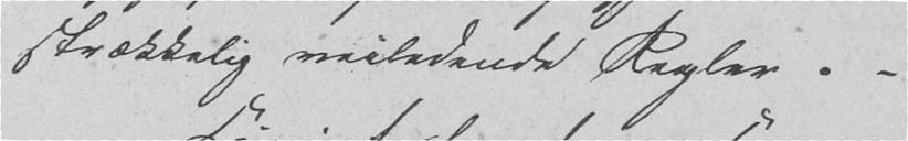strækkelig veiledende Regler. -
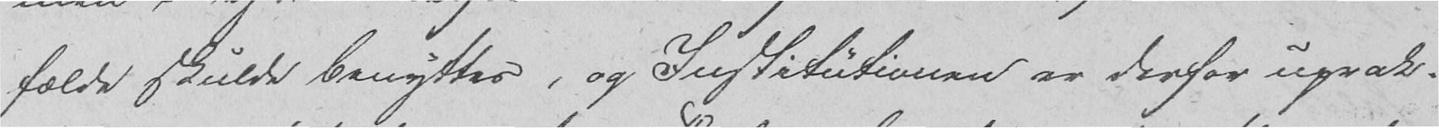fælde skulde benyttes, og Institutionen er derfor uprak-
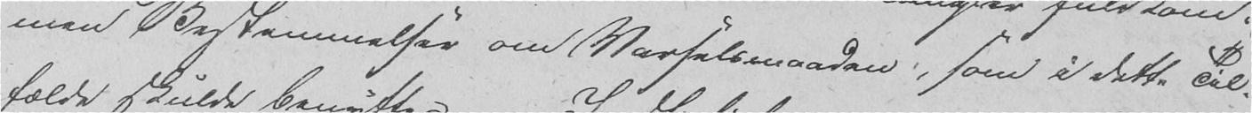men Bestemmelser om Varselsmaaden, som i dette Til-
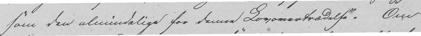som den almindelige for denne Lovovertrædelse. Om
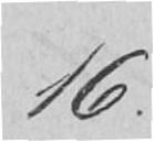16.
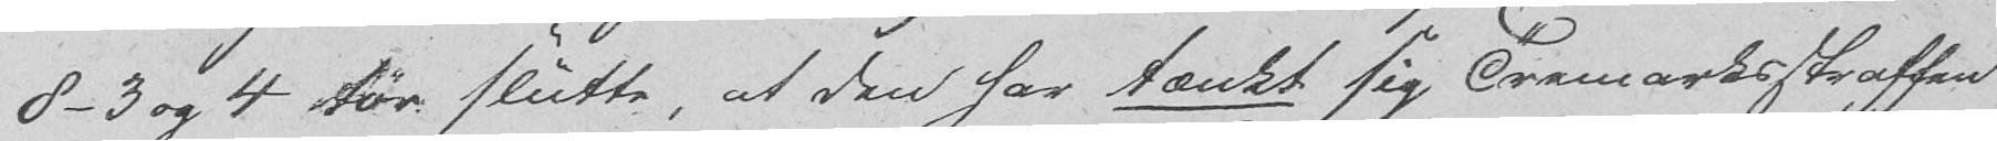8-3 og 4 tør slutte, at den har tænkt sig Tremarksstraffen
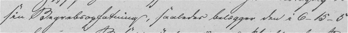sin Begrebsopfatning, saaledes belægger den i 6-15-5
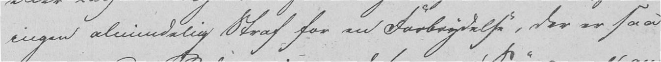ingen almindelig Straf for en Forbrydelse, der er saa
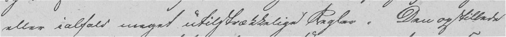eller ialfald meget utilstrækkelige Regler. Den opstillede
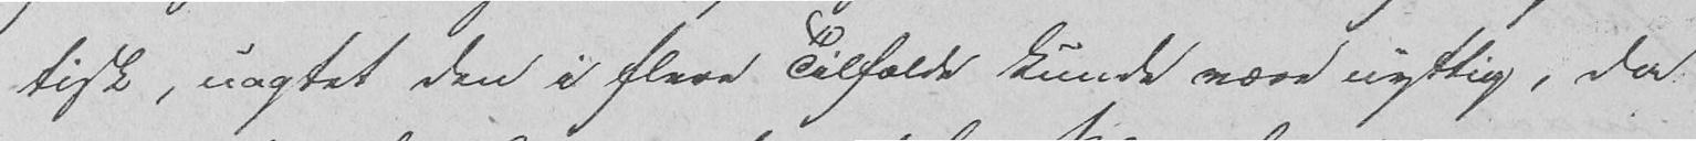tisk, uagtet den i flere Tilfælde kunde være nyttig, da
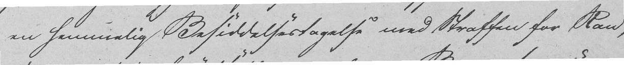en hemmelig Besiddelsestagelse med Straffen for Ran,
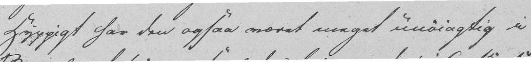Hyppigt har den ogsaa været meget unøiagtig i
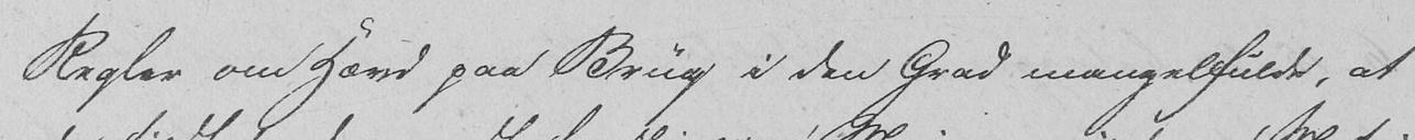Regler om Hævd paa Brug i den Grad mangelfulde, at
